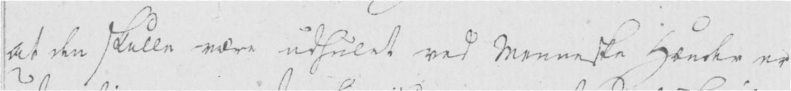at den skulle være udhulet ved Menneske Hænder er
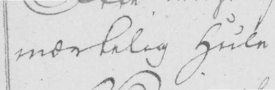mærkelig Hule
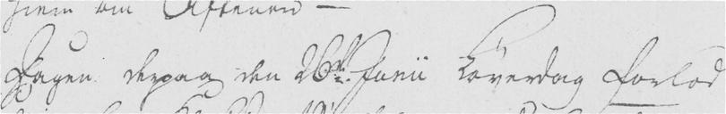Dagen derpaa den 26te Junii Løverdag forlod
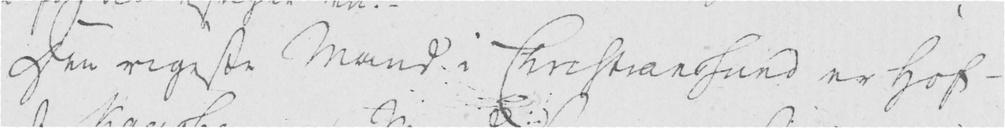Den rigeste Mand i Christianssund er Hof-
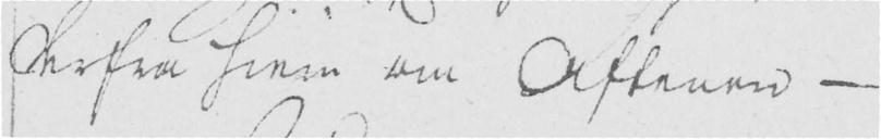derfra hiem om Aftenen -
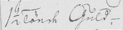1 1/2 Tønde Guld. -
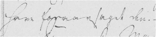have foraarsaget den. -
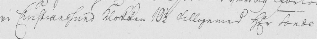vi Christianssund Klokken 10 1/2 tilligemed Hr sonde
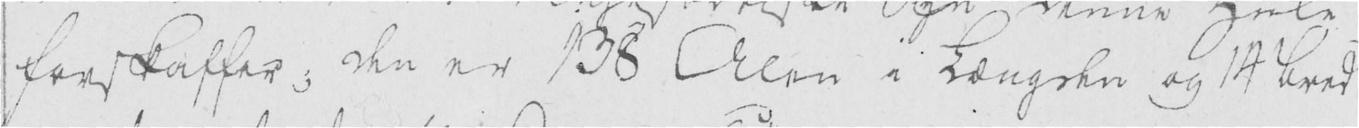forskaffer; den er 138 Alen i Længde og 14 bred
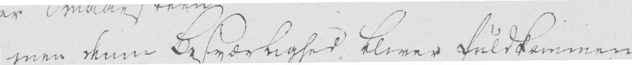men denne Besværlighed bliver fuldkommen
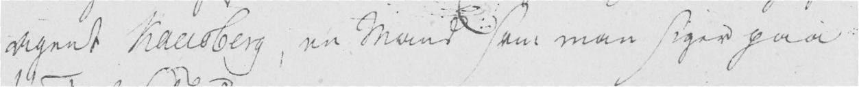agent Kaasberg, en Mand som man siger paa
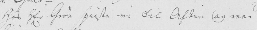Hos Hr Grøn spiiste vi til Aften og var
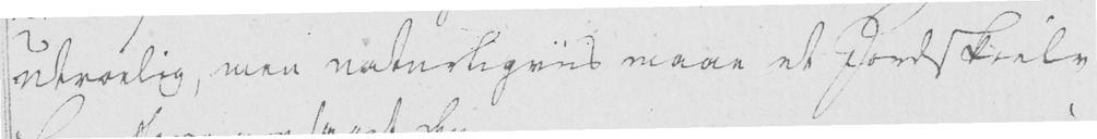utroelig, men naturligvis maae et Jordskielv
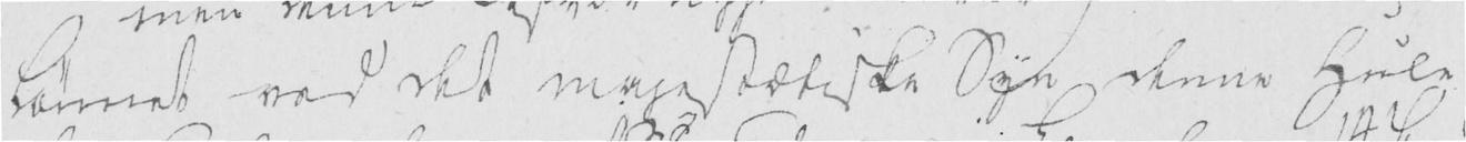bannet ved det majestætiske Syn denne Hule
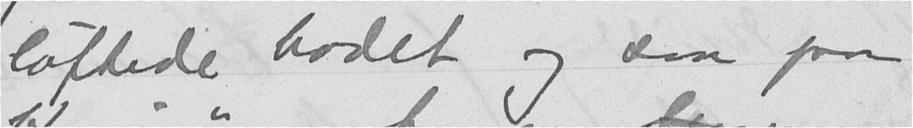løftede hodet og saa paa
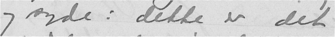og sagde: dette er det
